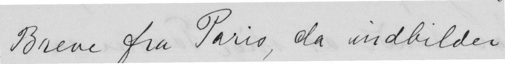Breve fra Paris, da indbilder
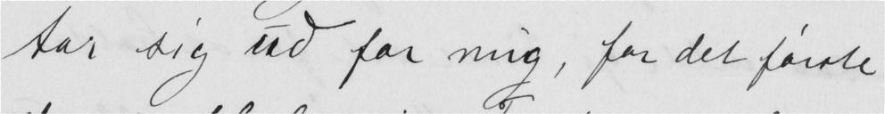tar sig ud for mig, for det første
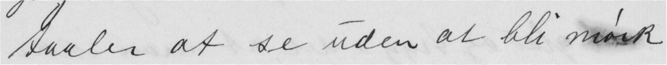taaler at se uden at bli mørk
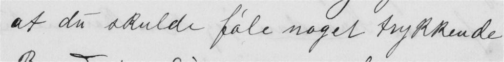at du skulde føle noget trykkende
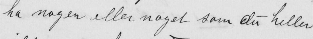ha nogen eller noget som du heller
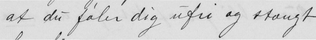at du føler dig ufri og stengt
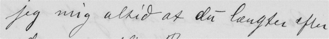jeg mig. altid at du længter efter
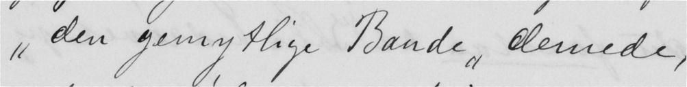den gemytlige Bande, dernede,
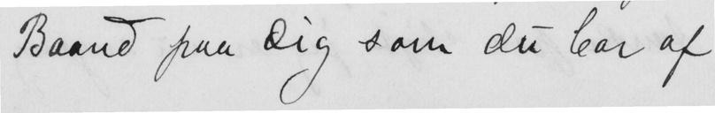Baand paa Dig som du bar af
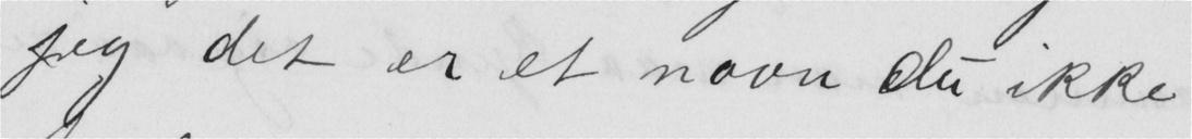jeg det er et navn du ikke
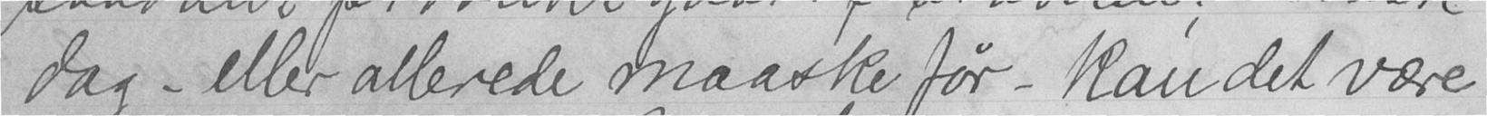dag - eller allerede maaske før - kan det være
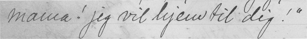mama! jeg vil hjem til dig!"
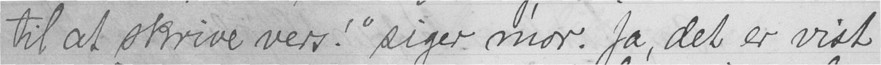til at skrive vers!" siger mor. Ja, det er vist
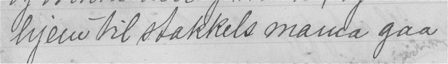hjem til stakkels mama gaa
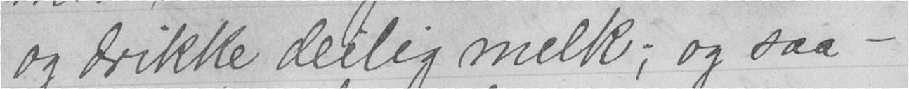og drikke deilig melk; og saa -
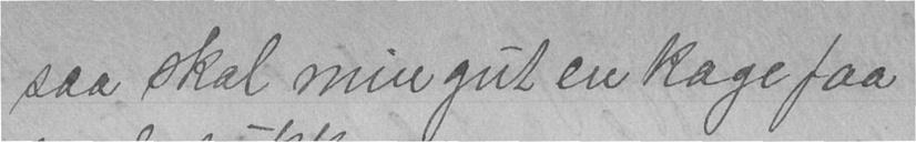saa skal min gut en kage faa
